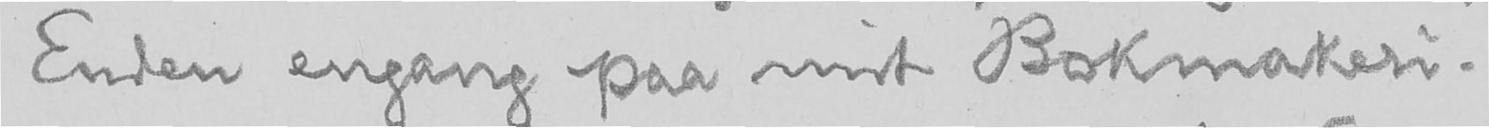Enden engang paa mit Bokmakeri.
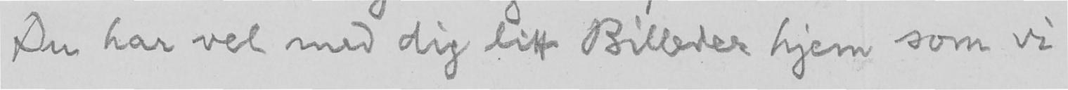Du har vel med dig litt Billeder hjem som vi
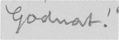Godnat!
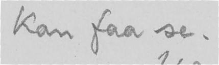kan faa se.
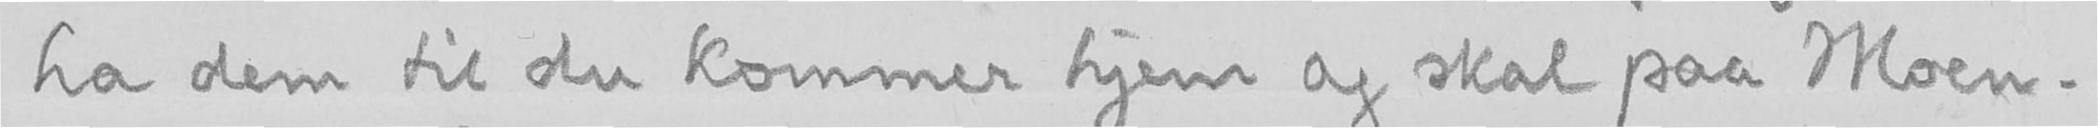ha dem til du kommer hjem og skal paa Moen.
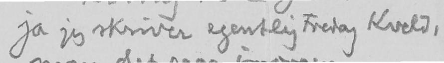ja jeg skriver egentlig Fredag Kveld,
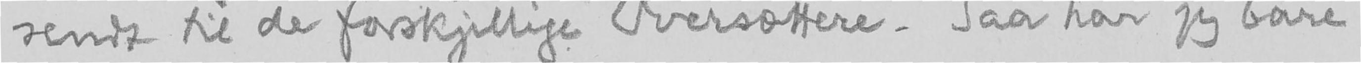sendt til de forskjellige Oversættere. Saa har jeg bare
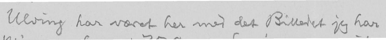Ulving har været her med det Billedet jeg har
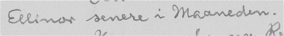Ellinor senere i Maaneden.
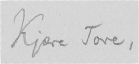Kjære Tore,
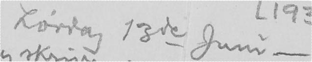Lørdag 13de Juni -
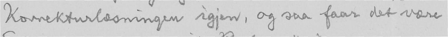Korrekturlæsningen igjen, og saa faar det være
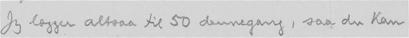Jeg lægger altsaa til 50 dennegang, saa du kan
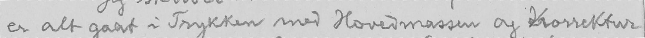er alt gaat i Trykken med Hovedmassen og Korrektur
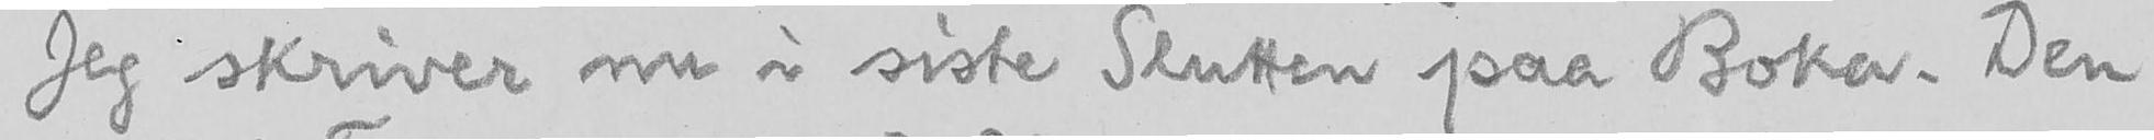Jeg skriver nu i siste Slutten paa Boka. Den
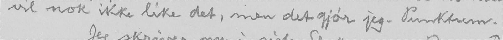vil nok ikke like det, men det gjør jeg. Punktum.
